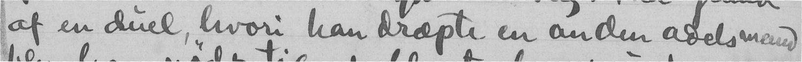af en duel, hvori han dræpte en anden adelsmand
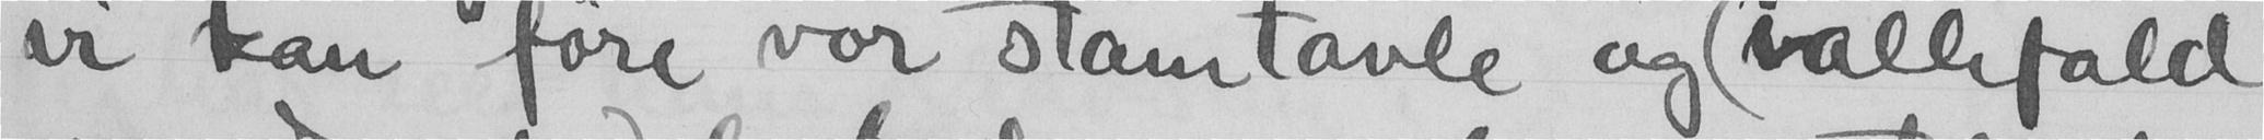vi kan føre vor stamtavle og (iallefald
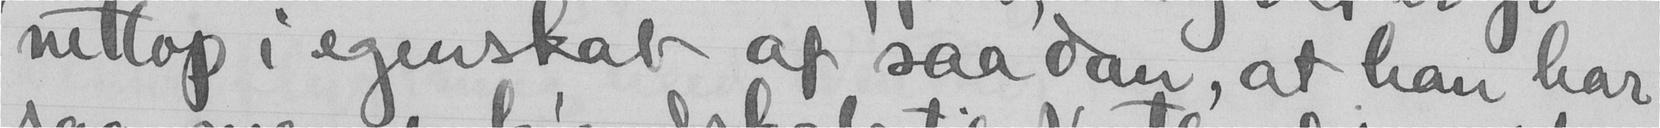nettop i egenskab af saadan, at han har
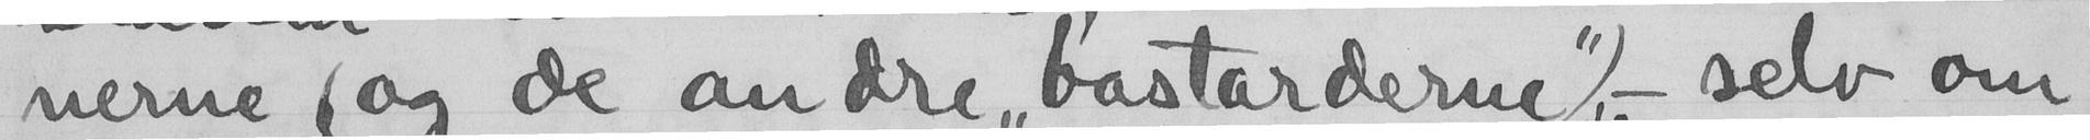nerne (og de andre "bastarderne" - selv om
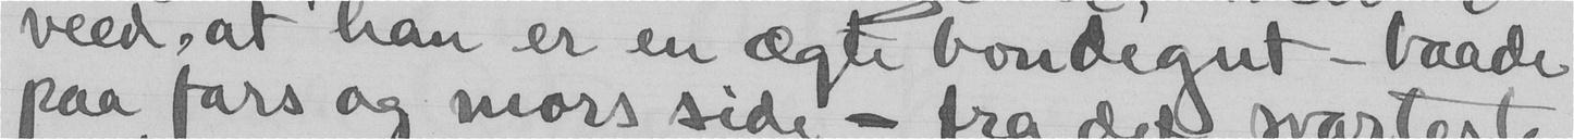veed, at han er en ægte bondegut - baade
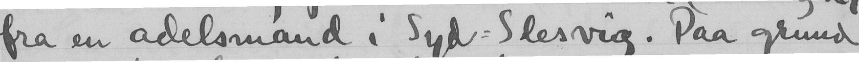fra en adelsmand i Syd-Slesvig. Paa grund
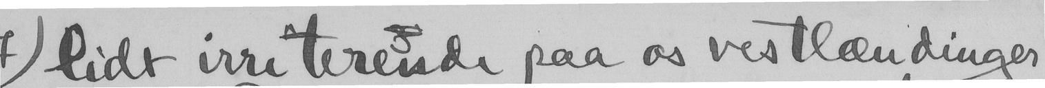lidt irreterende paa os vestlændinger
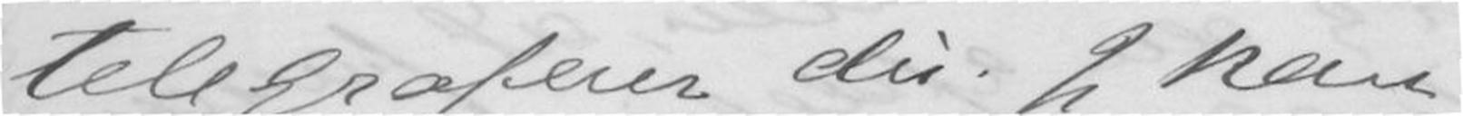telegraferer du. Jeg kan
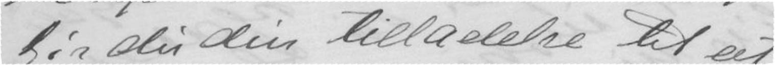Gir du din tilladelse til at
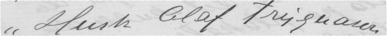"Husk Olaf Trygvason"
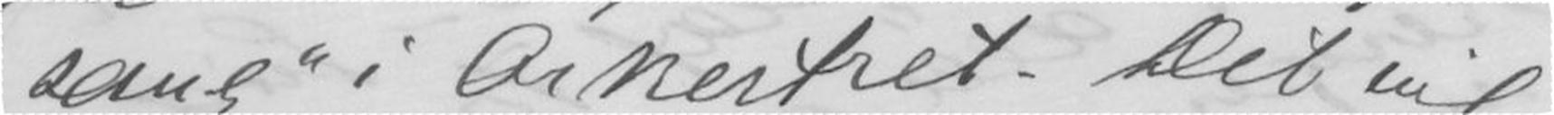sang i Orkestret. Det vil
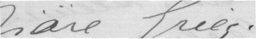Kjære Grieg!
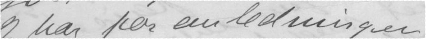Jeg har for anledningen
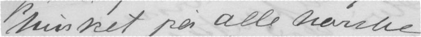husket på alle norske
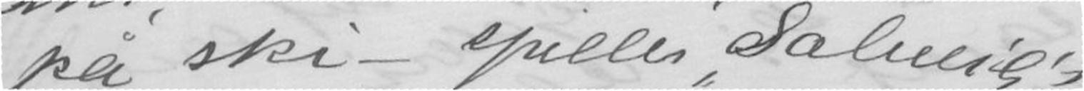på ski - spilles Solveig's
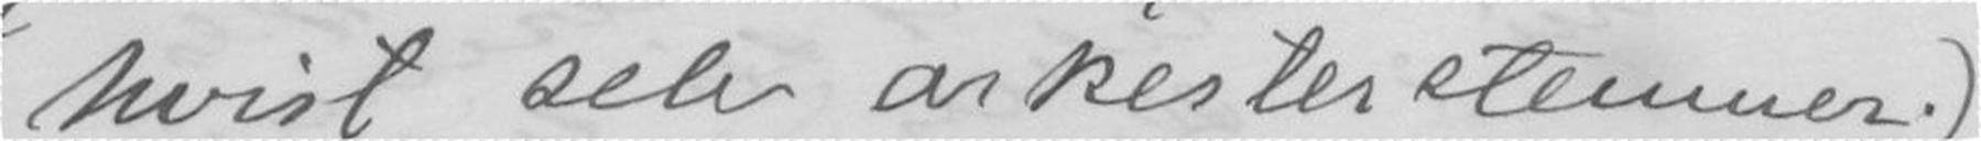hvist selv orkesterstemmer.)
